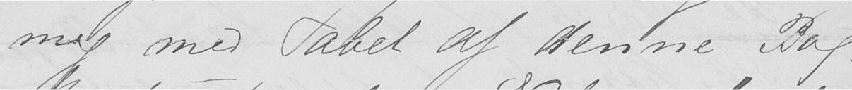mig med Tabet af denne Bog.
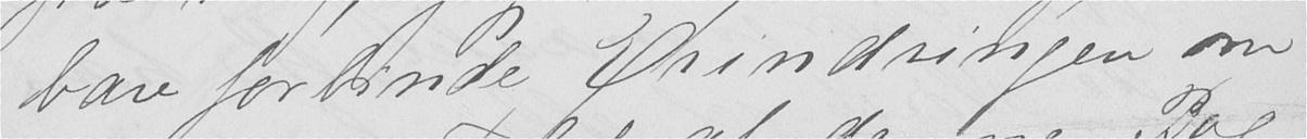bare forbinde Erindringen om
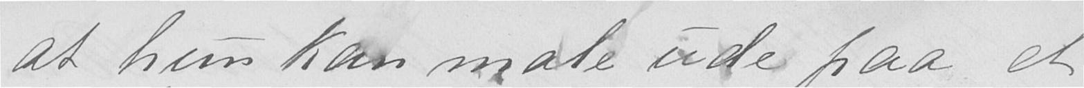at hun kan male ude paa et
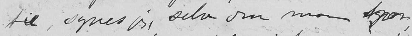til, synes jeg, selv om man tror
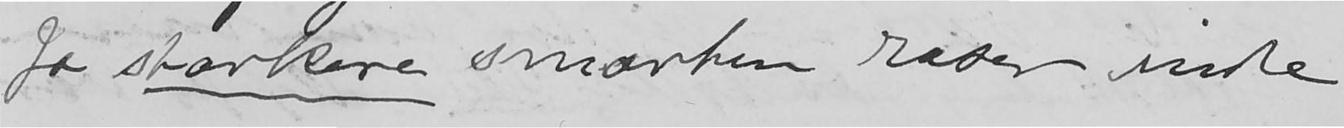Jo stærkere smærten raser inde
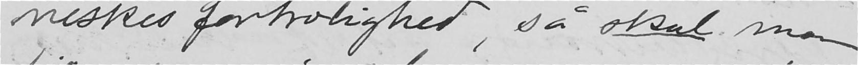neskes fortrolighed, så skal man
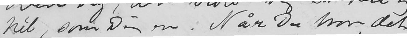hél, som Du er. Når Du tror det
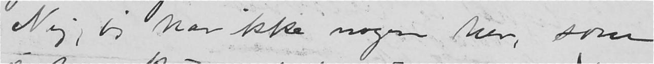Nej, jeg har ikke nogen her, som
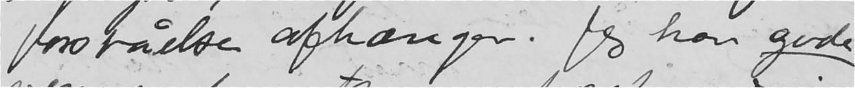forståelse afhænger. Jeg har gode
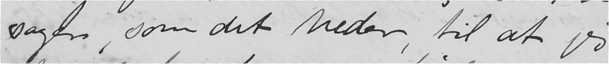sager, som det heder, til at jeg
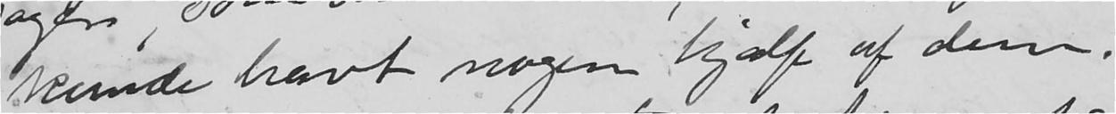kunde havt nogen hjælp af dem.
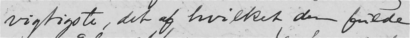vigtigste, det af hvilket den fulde
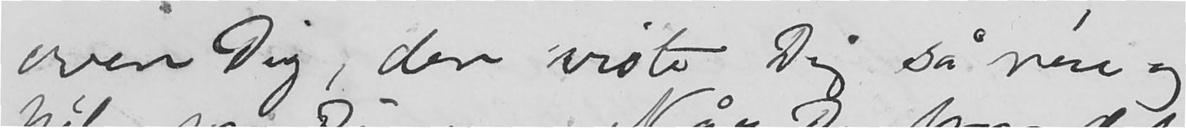over Dig, der viste Dig så rén og
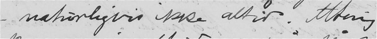- naturligvis ikke altid. Alting
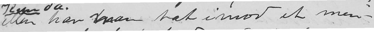Men har man tat imod et men-
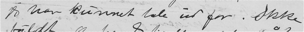jeg har kunnet tale ud for. Ikke
